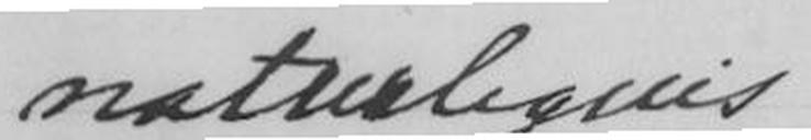naturligvis
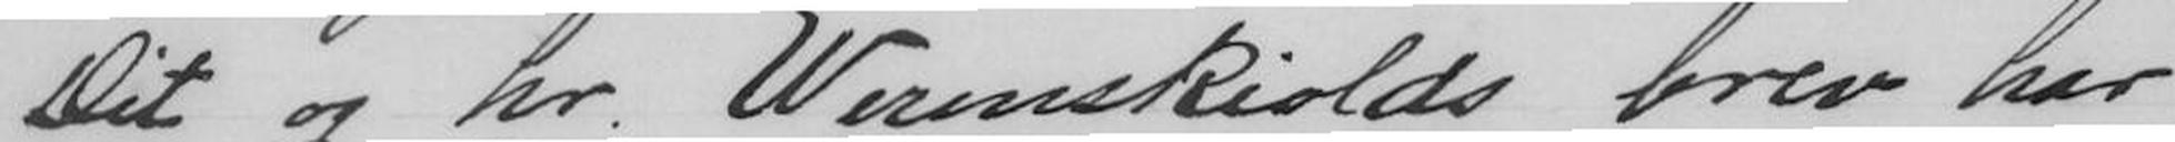Dit og hr. Wernskiolds brev har
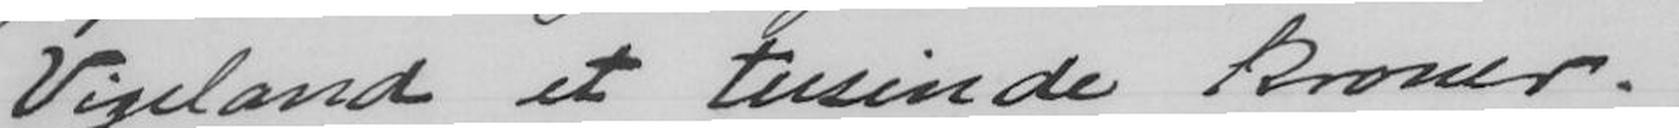Vigeland et tusinde kroner.
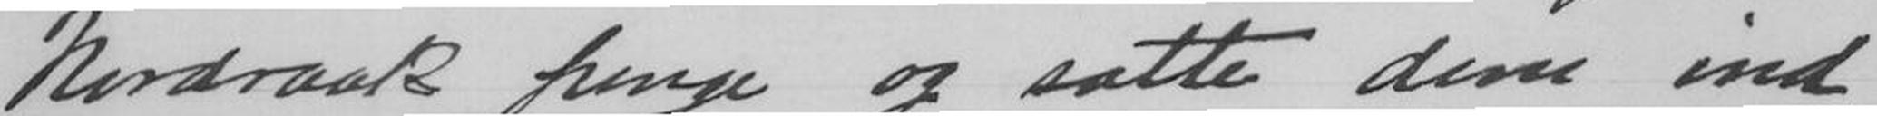Nordraak penge og satte dem ind
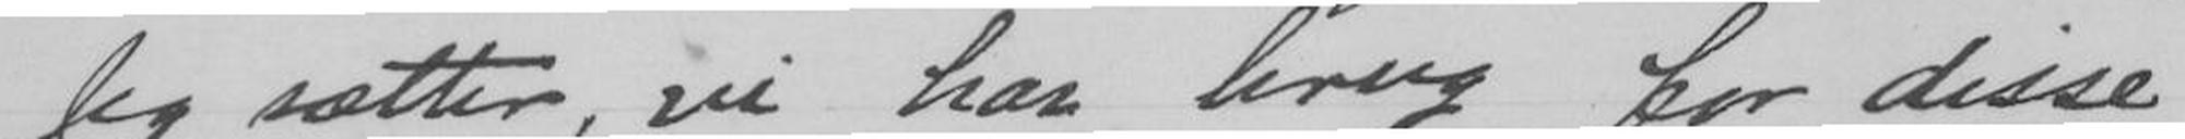Jeg sætter, vi har brug for disse
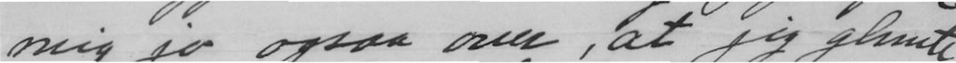mig jo ogsaa over, at jeg glemte
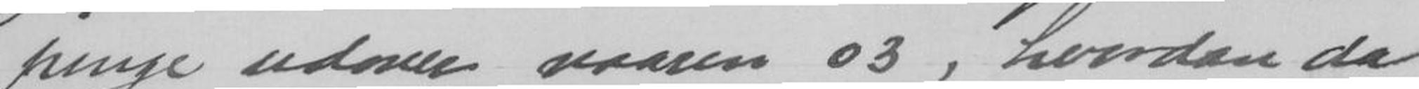penge udover vaaren 03, hvordan da
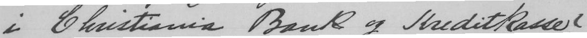i Christiania Bank og Kreditkasse?
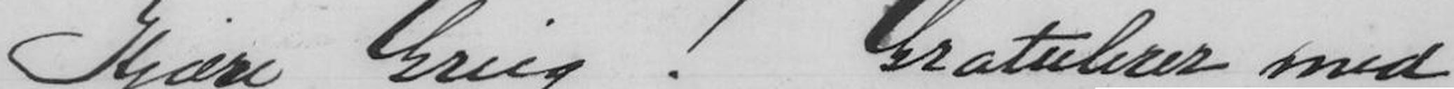Kjære Grieg! Gratulerer med
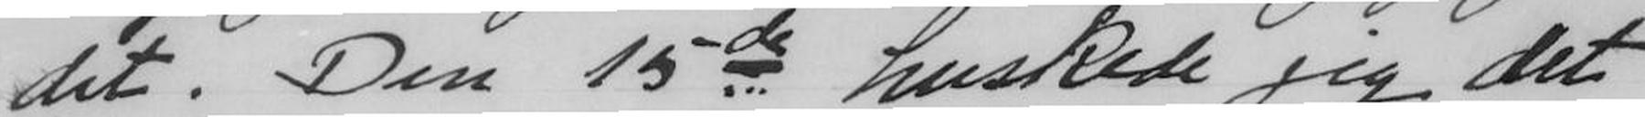det. Den 15de huskede jeg det
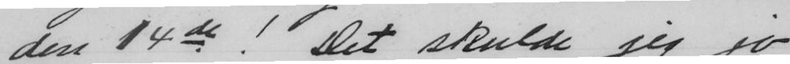den 14de! Det skulde jeg jo
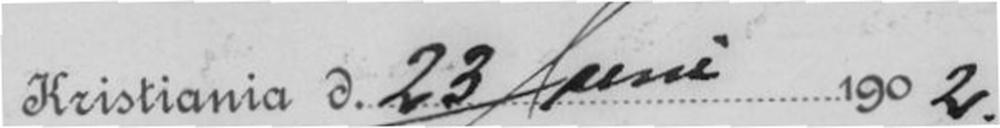Kristiania d. 23 Juni 1902.
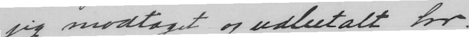jeg modtaget og udbetalt hr.
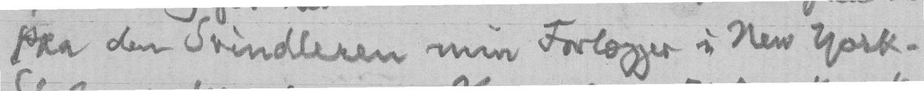paa den Svindleren min Forlægger i New York.
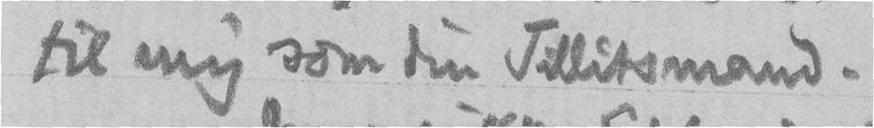til mig som din Tillitsmand.
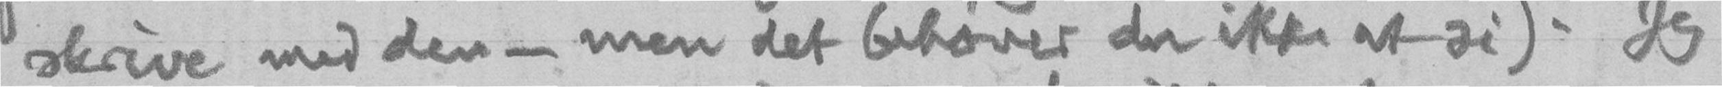skrive med den - men det behøver du ikke at si). Jeg
